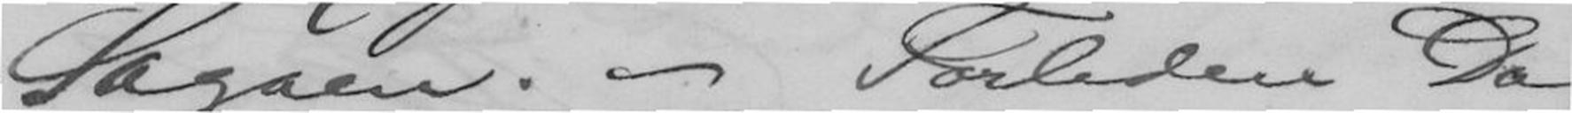Sagaen. - Forleden Dag
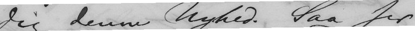Dig denne Nyhed. Saa ser
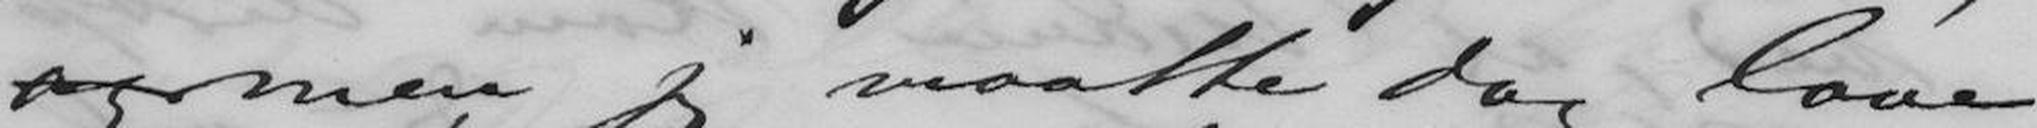og men jeg maatte dog love
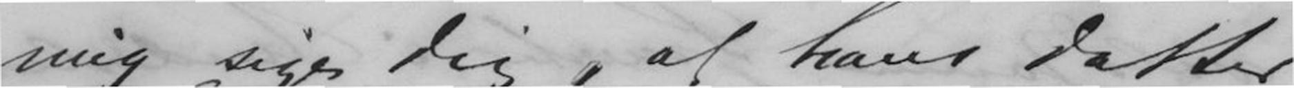mig sige dig, at hans Datter
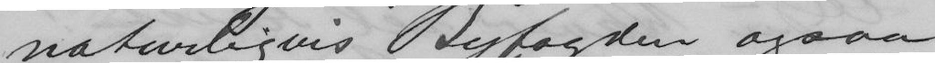naturligvis Byfogden ogsaa
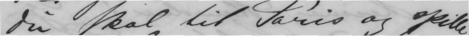Du skal til Paris og spille
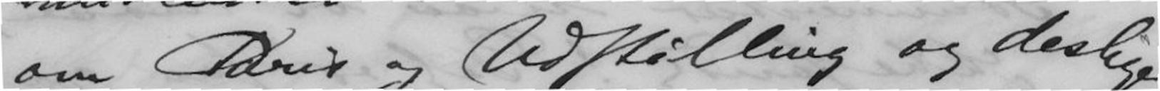om Paris og Udstilling og deslige,
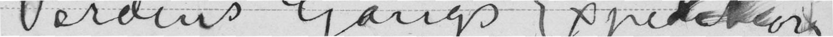Verdens Gangs Expedition
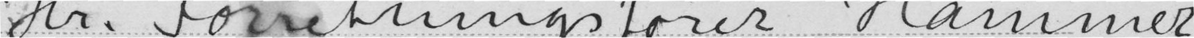Hr. Forretningsfører Hammer
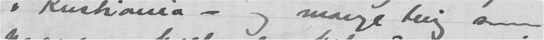i Kristiania - og mange ting som
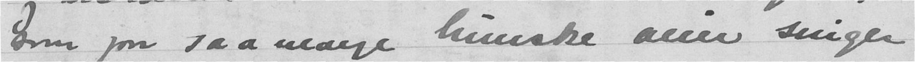som paa saa mange lumske veier sniger
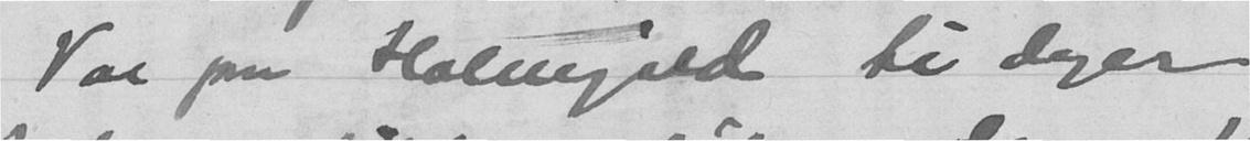Var paa Holmgild ti dager
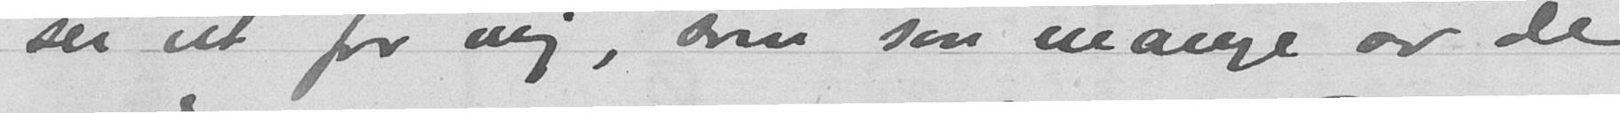ser ut for mig, som saa mange av de
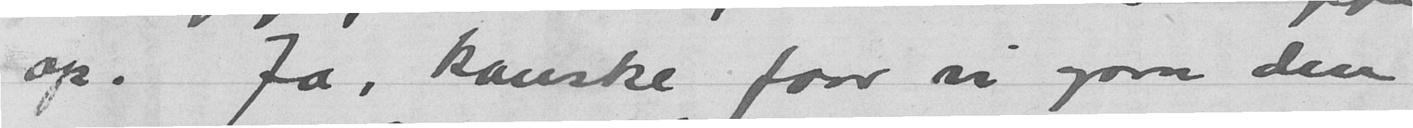op. Ja, kanske faar vi ogsaa den
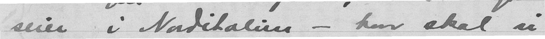seier i Norditalien - hvor skal vi
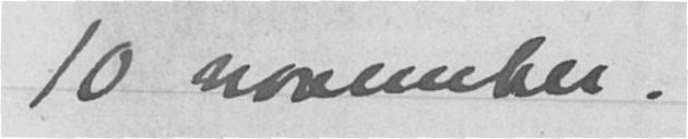10 november.
